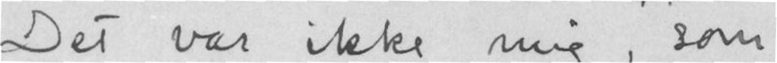Det var ikke mig, som
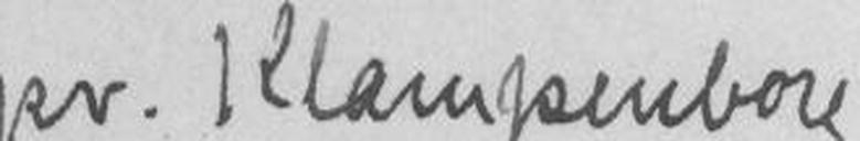pr. Klampenborg
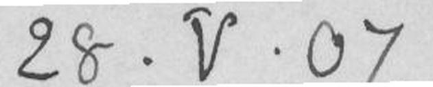28.V.07
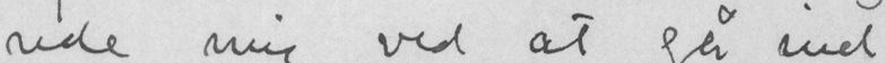rede mig at gå ind
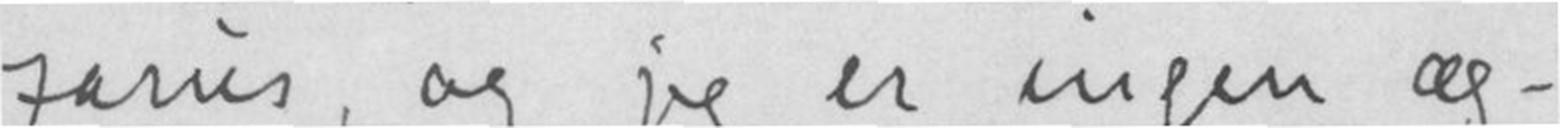zarus, og jeg er ingen æg-
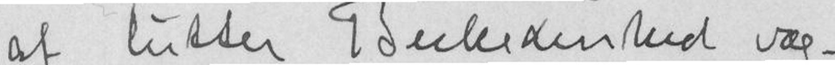af lutter Beskedenhed væg-
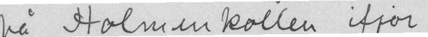på Holmenkollen ifjor
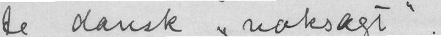te dansk "noksagt".
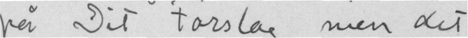på Dit Forslag, men det
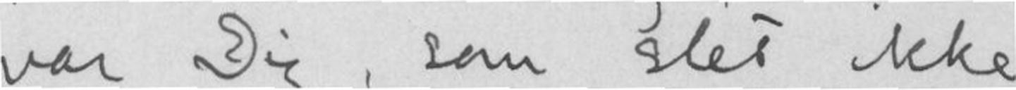var Dig, som slet ikke
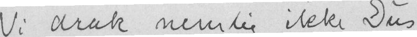Vi drak nemlig ikke Dus
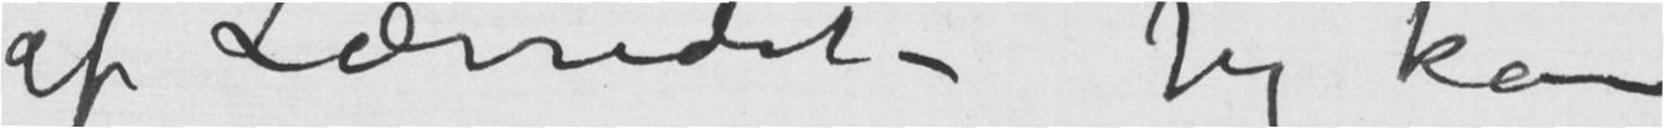af Lærredet – Jeg kan
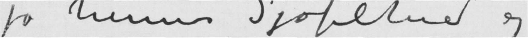jo hennes Sjofelthed og
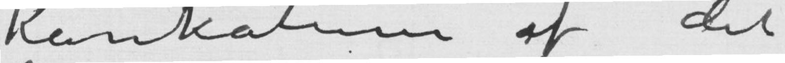Karikaturen af det
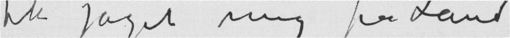fik jaged mig fra Land
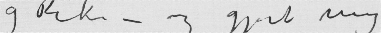og Rike – og gjort mig
